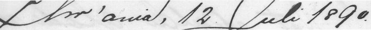Chr'ania, 12 Juli 1890.
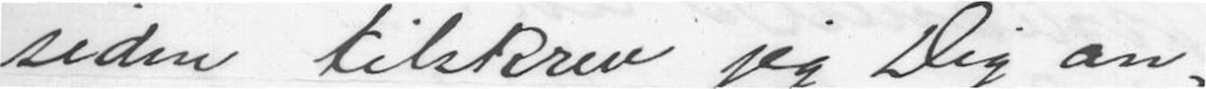siden tilskrev jeg Dig an-
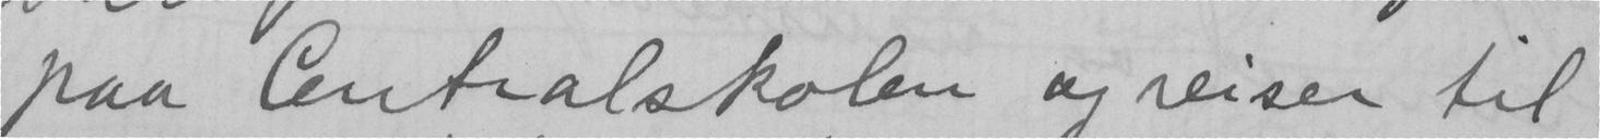paa Centralskolen og reiser til
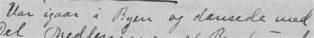Var igaar i Byen og dansede med
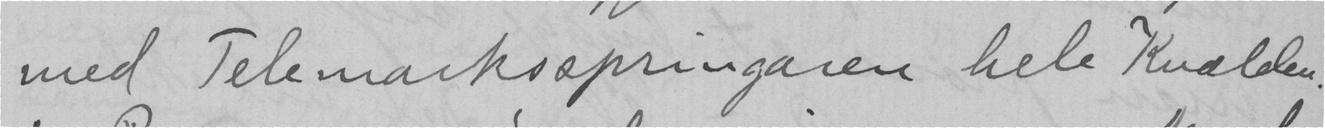med Telemarksspringaren hele kvælden.
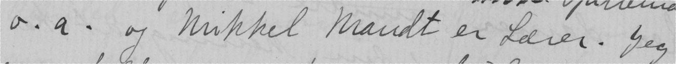a. a. og Mikkel Mandt er Lærer. Jeg
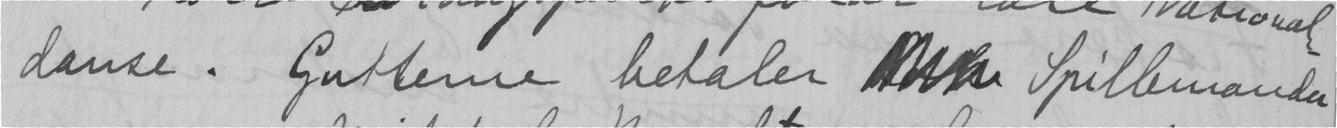danse. Gutterne betaler  Spillemanden
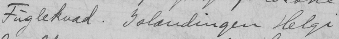Fuglekvad. Islændingen Helgi
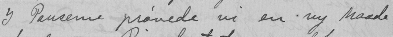I Panserne prøvede vi en ny Maade
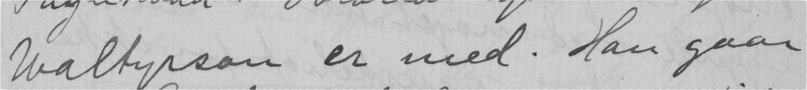Waltyrson er med. Han gaar
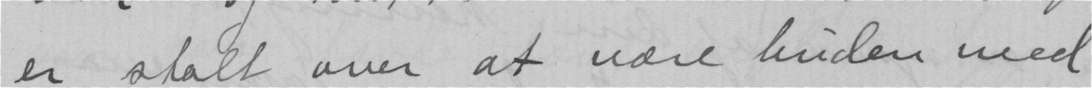er stolt over at være buden med
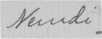Nemdi
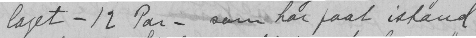laget -12 Par - som har faat istand
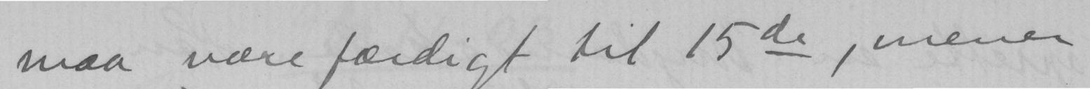maa være færdigt til 15de, mener
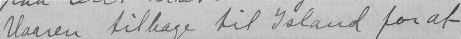Vaaren tilbage til Island for at
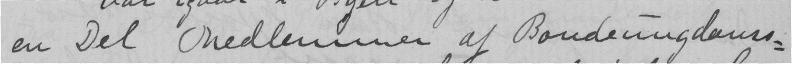en Del Medlemmer af Bondeungdoms-
